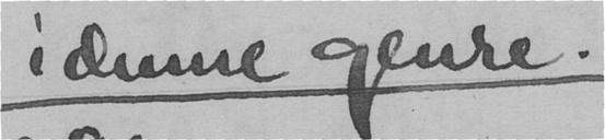i denne genre.
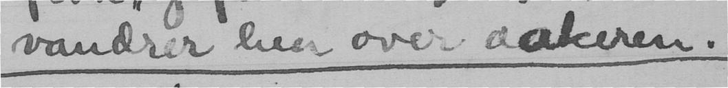vandrer hen ove aakeren.
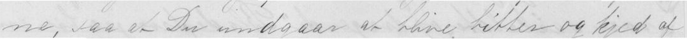ne, saa at den indgaar at blive bitter og kjed af
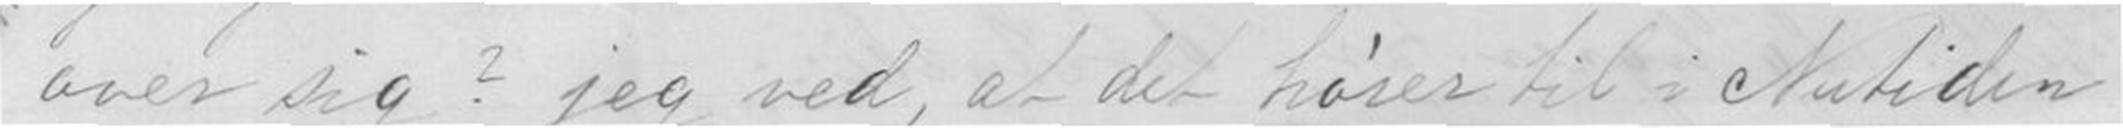over sig? jeg ved, at det hører til i Nutiden.
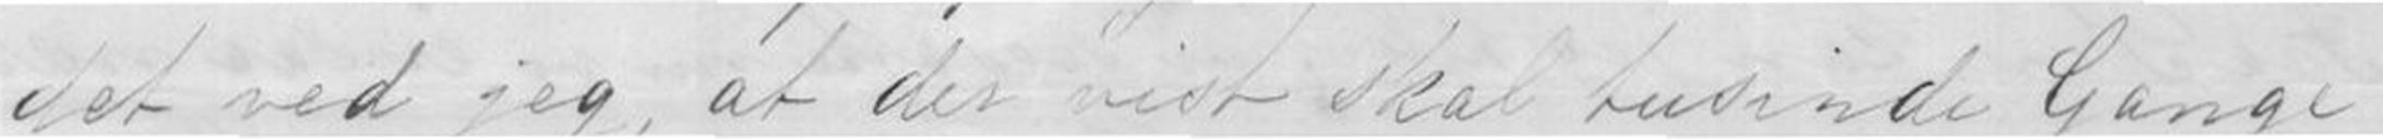det ved jeg, at der vist skal tusinde Gange
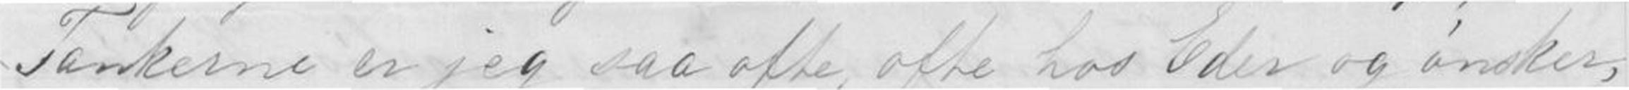Tankerne er jeg saa ofte, ofte hos Eder og ønsker,
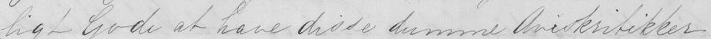ligt Gode at have disse dumme Aviskritikker
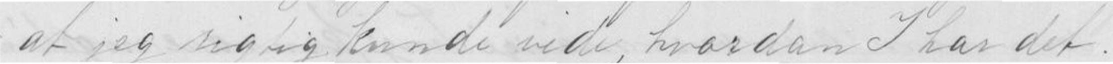at jeg rigtig kunde vide, hvordan I har det.
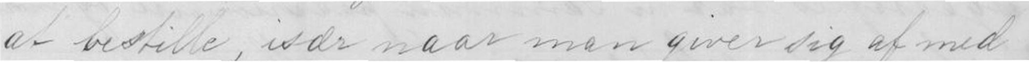at bestille, især naar man giver sig af med
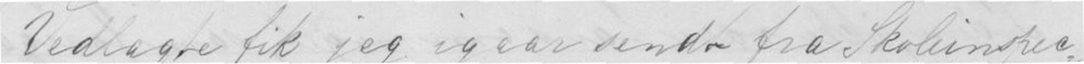Vedlagte fik jeg igaar sendt fra Skoleinspec-
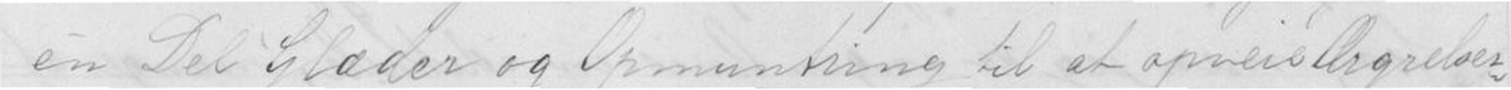én Del Glæder og Opmuntring til at opveie Ærgrelser-
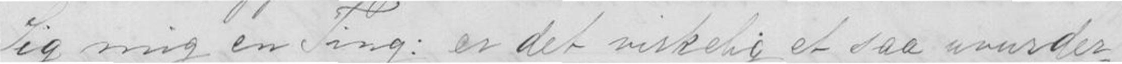Sig mig en Ting: er det virkelig et saa uvurder-
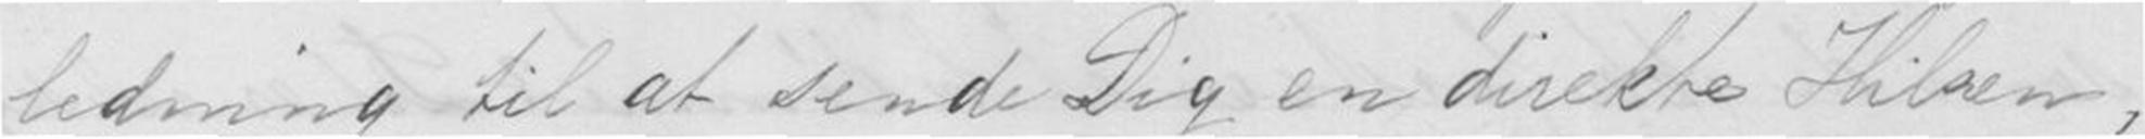ledning til at sende Dig en direkte Hilsen,
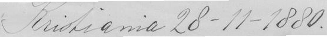Kristiania 28-11-1880
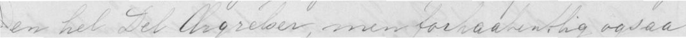en hel Del Ærgrelser, men forhaabentlig ogsaa
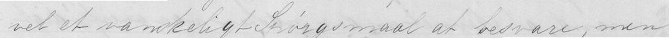vel et vanskeligt Spørgsmaal at besvare, men
